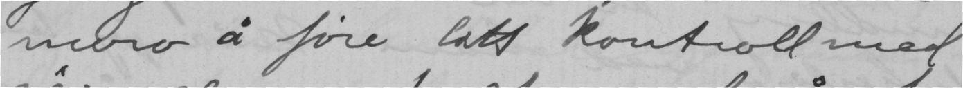moro å føre litt kontroll med
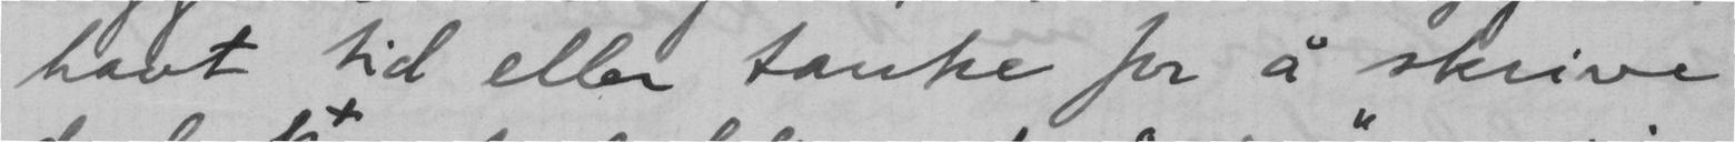havt tid eller tanke for å skrive
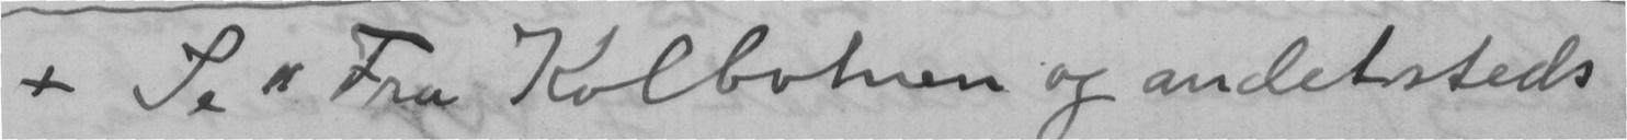x Se "Fra Kolbotnen og andetsteds
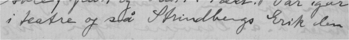i teatre og så Strindbergs Erik den
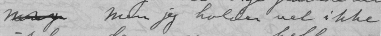Men jeg holder vel ikke
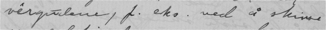vêrgudene, f. eks. ved å skive
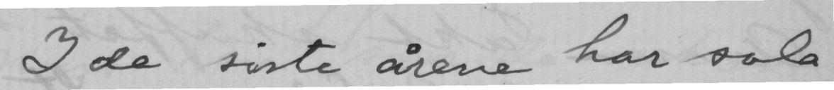I de siste årene har sola
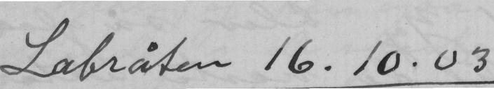Labråten 16.10.03
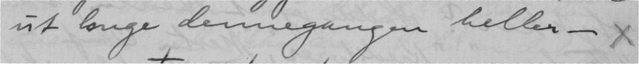ut længe dennegangen heller - x
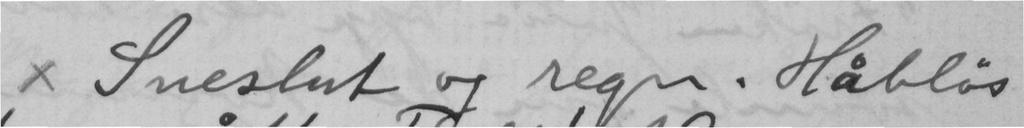x Sneslut og regn. Håbløs
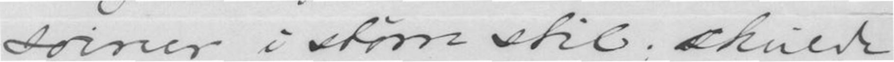soireer i større stil; skulde
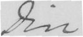Din
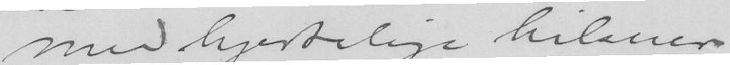Med hjertelige hilsener
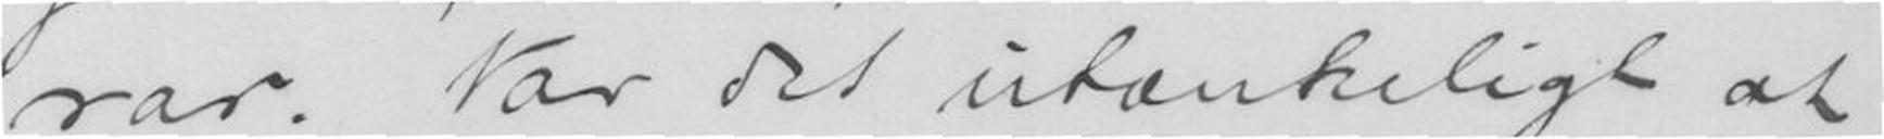rar. Var det utænkeligt at
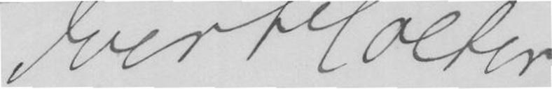Iver Holter
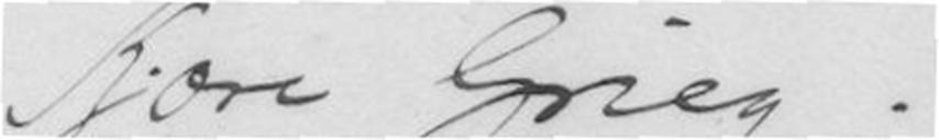Kjære Grieg!
